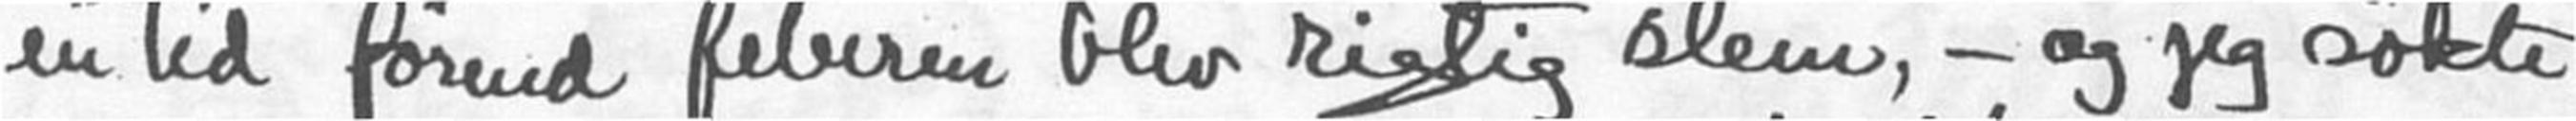en tid førend feberen blev rigtig slem, - og jeg søkte
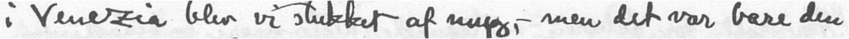i Venezia blev vi stukket af myg, - men det var bare den
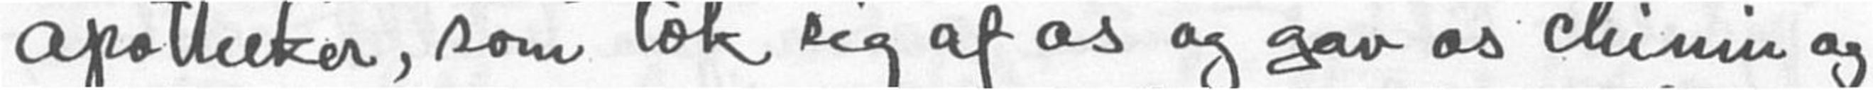apotheker, som tok sig av oss og gav os chinin og
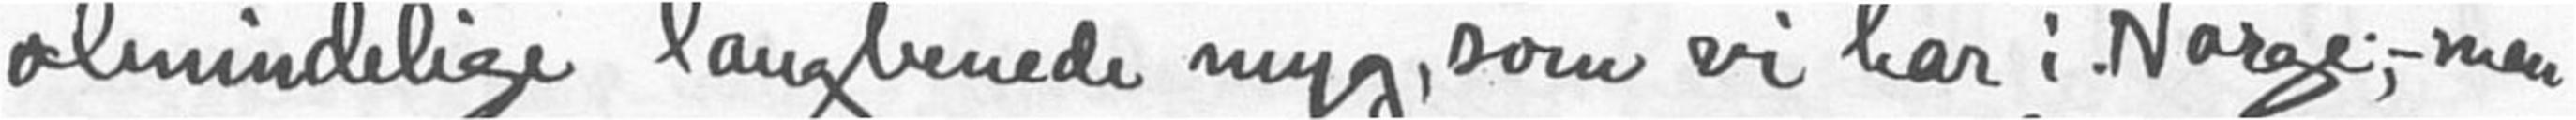almindelige langbenede myg, som vi har i Norge; - men
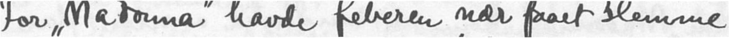For "Madonna" havde feberen nær faaet slemme
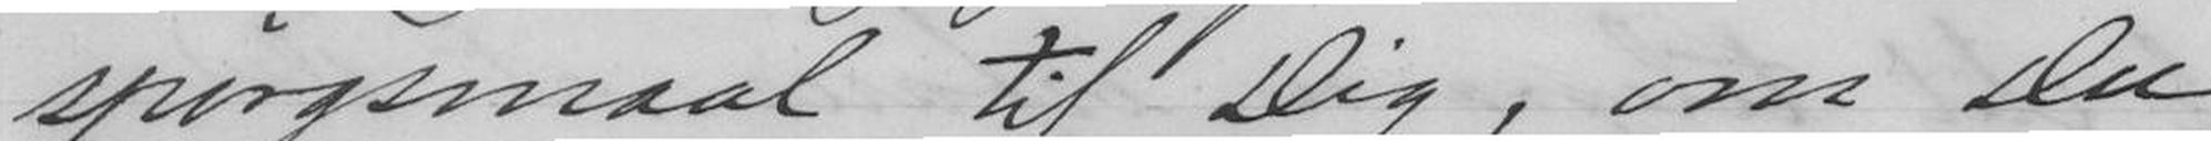spørgsmaal til Dig, om Du
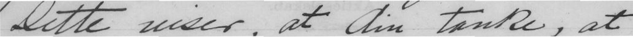Dette viser, at din tanke, at
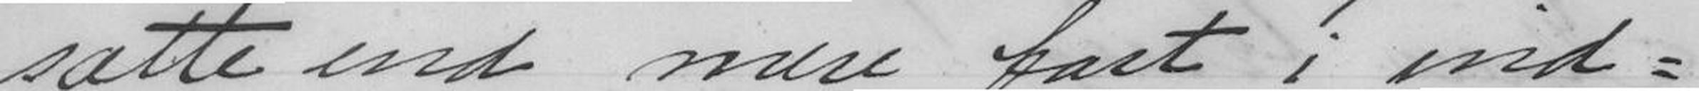sætte end mere fart i ind=
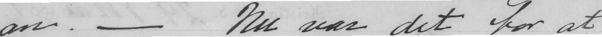an. - Nu var det for at
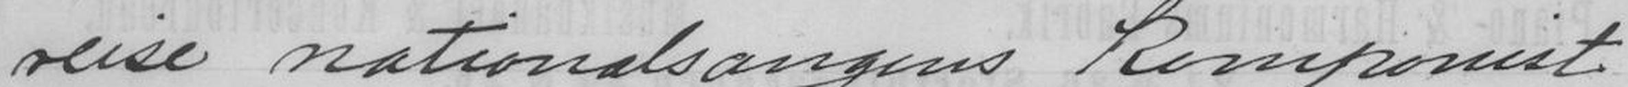reise nationalsangens komponist
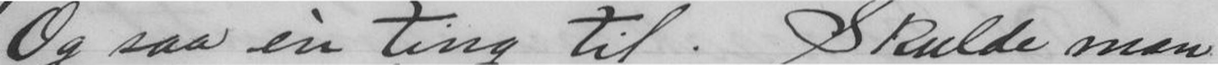Og saa èn ting til. Skulde man
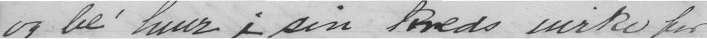og be' hver i sin kreds virke for
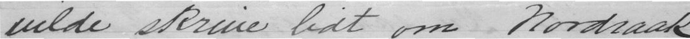vilde skrive lidt om Nordraak
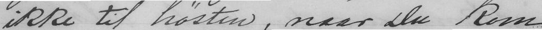ikke til høsten, naar Du kom
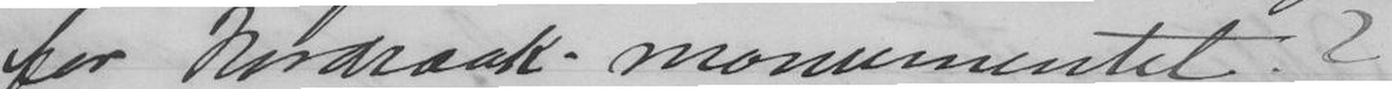for Nordraak- monumentet?
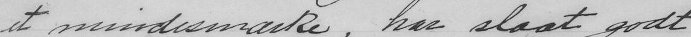et mindesmærke, har slaat godt
